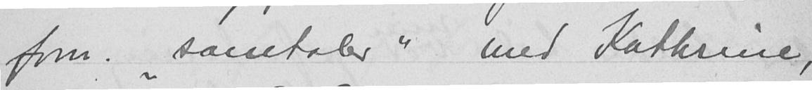form. "samtaler" med Kathrine,
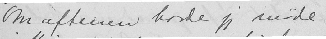Om aftenen havde jeg møde
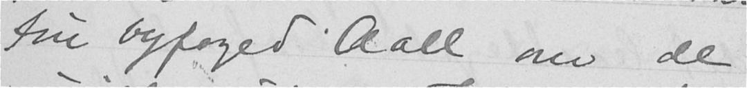fru byfoged Aall om de
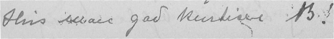Hvis man gad kurtisere B!
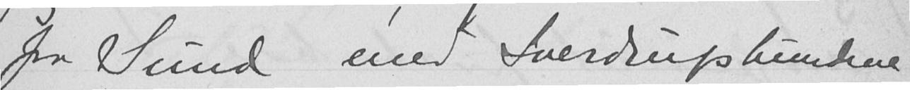fra Sund med Sverdrupshundene
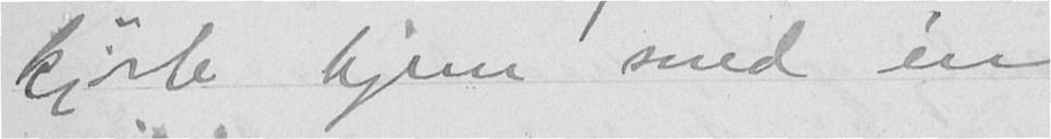kjørte hjem med én
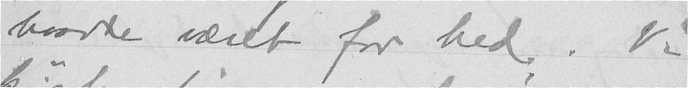havde været for hed. Vi
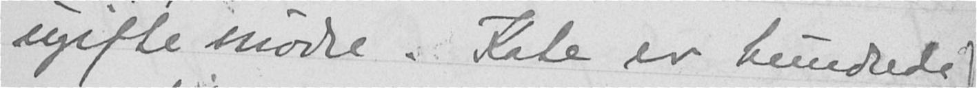ugifte mødre. Kate er hundrede
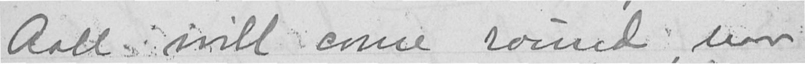Aall will come round, naar
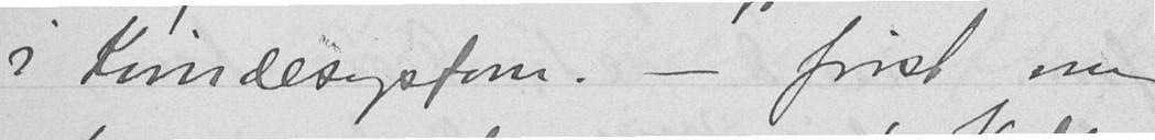i Kvindesagsforn. - først om
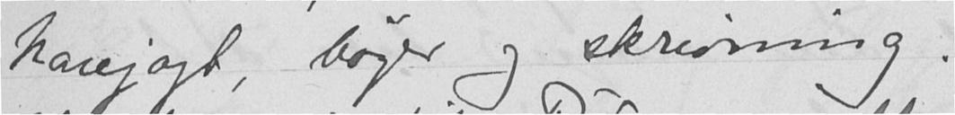harejagt, bøger og skrivning.
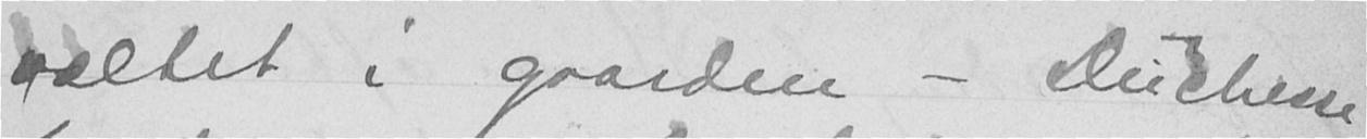væltet i gaarden - Duchesse
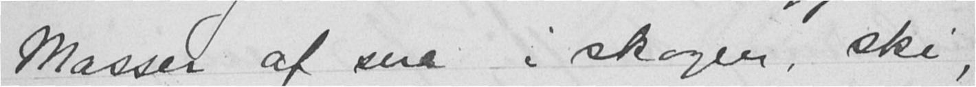Masser af sne i skogen, ski,
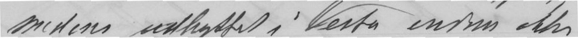medens udbyttet i Veste endnu ikke
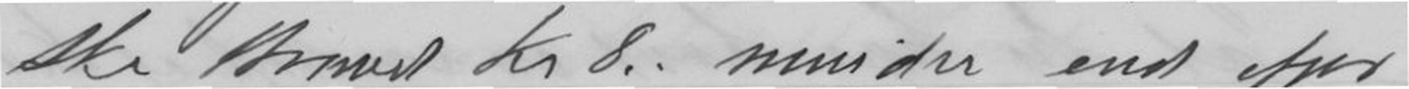ske Brand kr 8. mindre end ifjord,
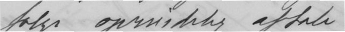følge oprindelig aftale.
